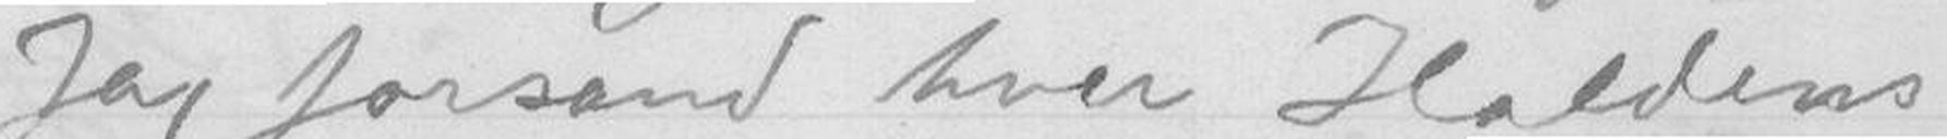Ja, forsand hver Haldens
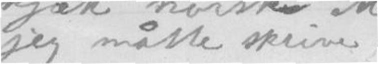jeg måtte skrive
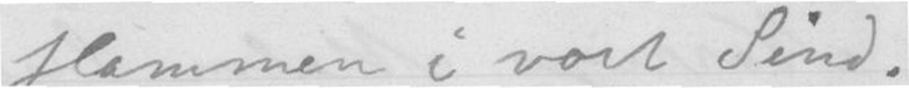flammer i vort Sind.
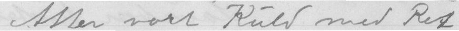Atter vort Kuld med Ret
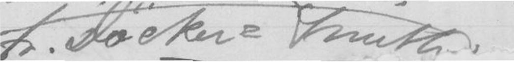Fr. Döcker=Smith
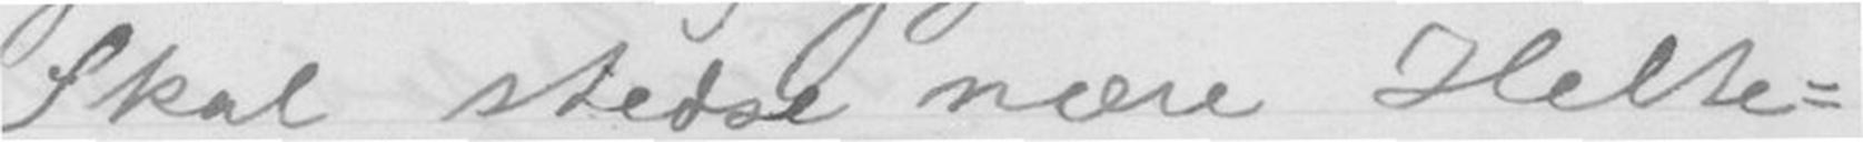Skal stedse nære Helte-
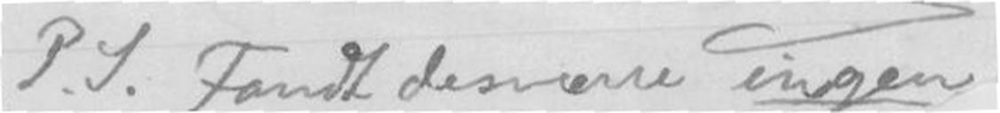P.S. Fandt desværre ingen
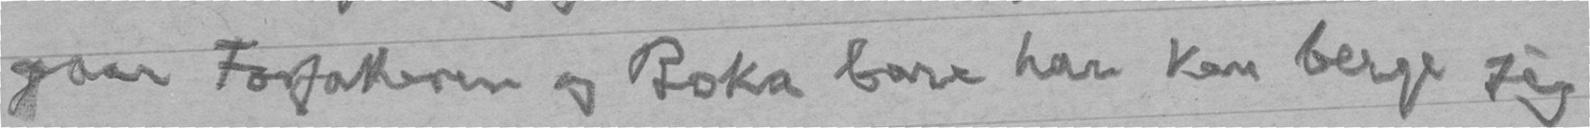gaar Forfatteren og Boka bare han kan berge sig
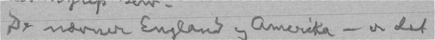De nævner England og Amerika - er det
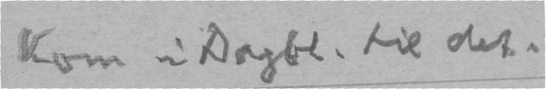kom i Dagbl. til det.
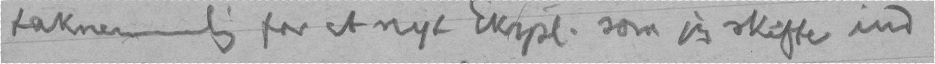taknemmelig for et nyt Ekspl. som jeg skifte ind
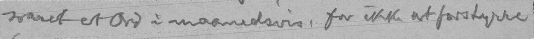svaret et Ord i maanedsvis, for ikke at forstyrre
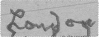London
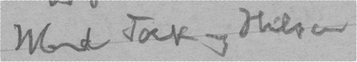Med Tak og Hilsen
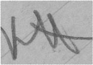KH
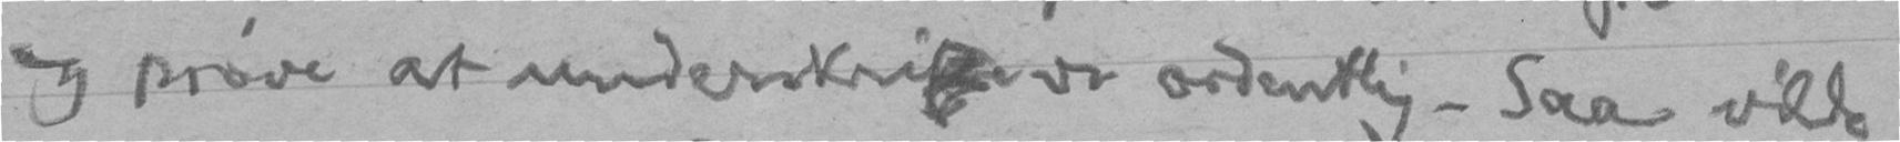og prøve at underskrive vi ordentlig. Saa vilde
